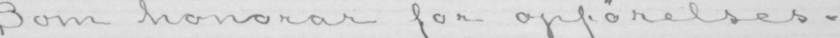Som honorar for opførelses-
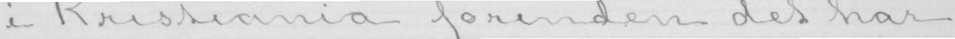i Kristiania forinden det har
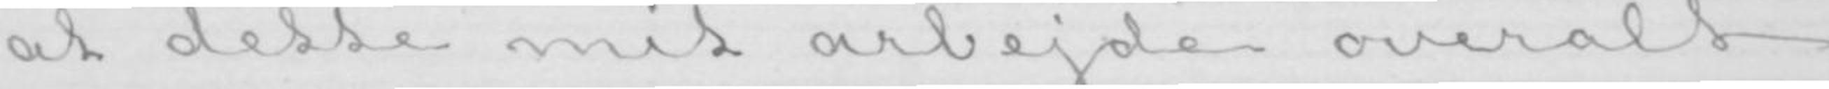at dette mit arbejde overalt
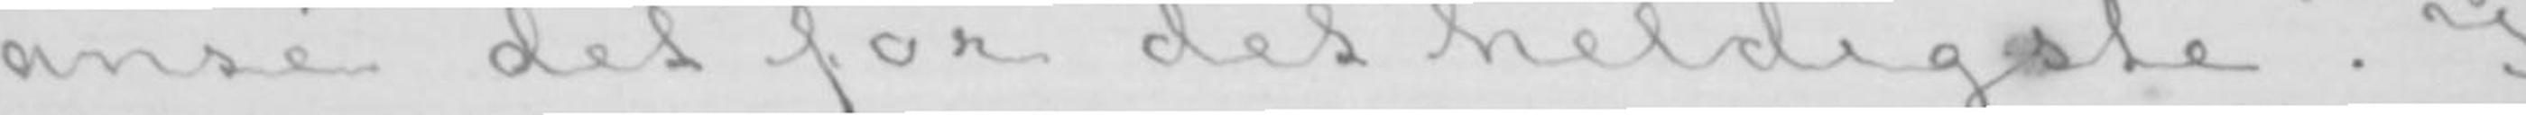ansé det for det heldigste. I
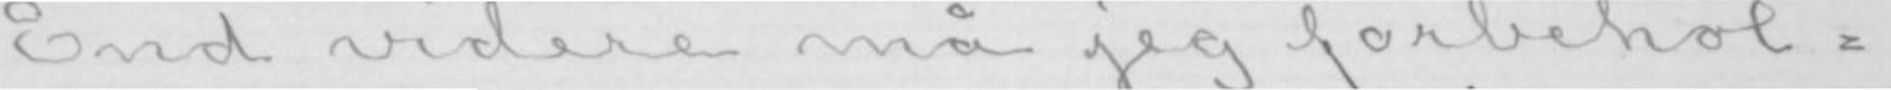End videre må jeg forbehol-
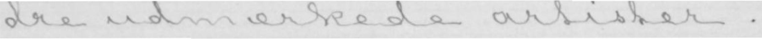dre udmærkede artister.
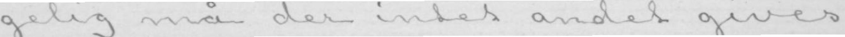gelig må der intet andet gives
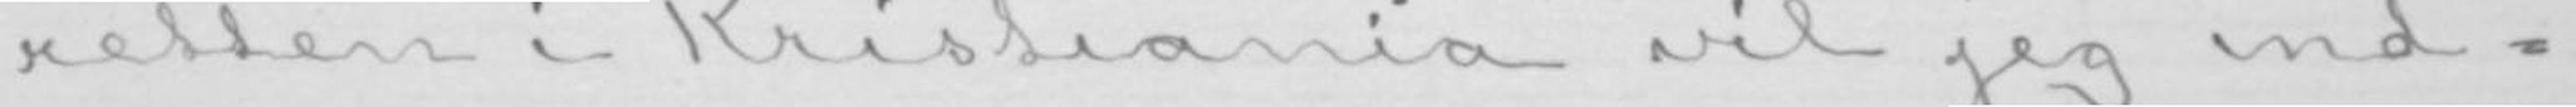retten i Kristiania vil jeg ind-
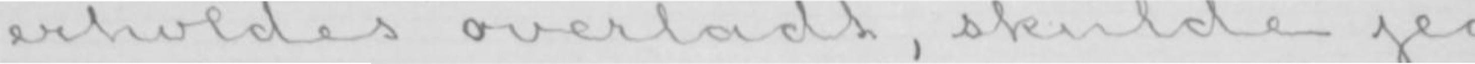erholdes overladt, skulde jeg
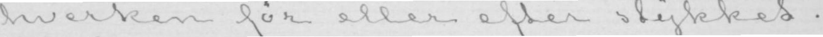hverken før eller efter stykket.
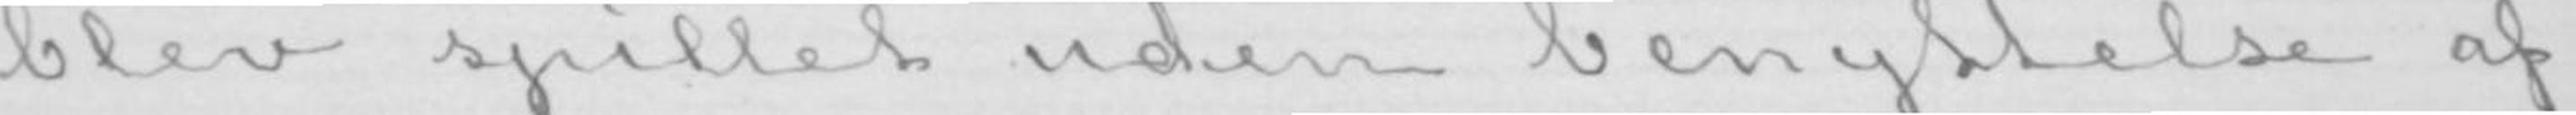blev spillet uden benyttelse af
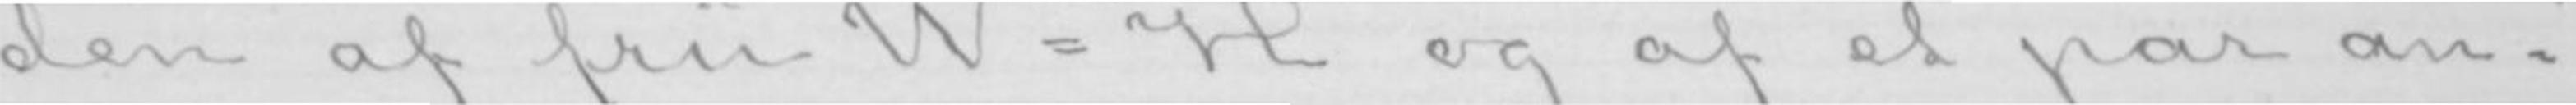den af fru W-H og af et par an-
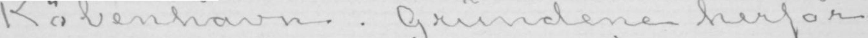København. Grundene herfor
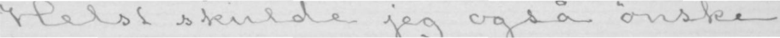Helst skulde jeg også ønske
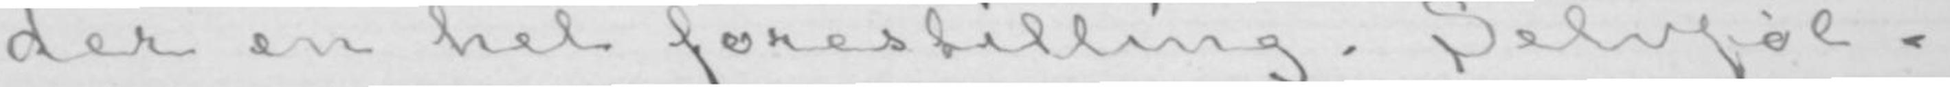der en hel forestilling. Selvføl-
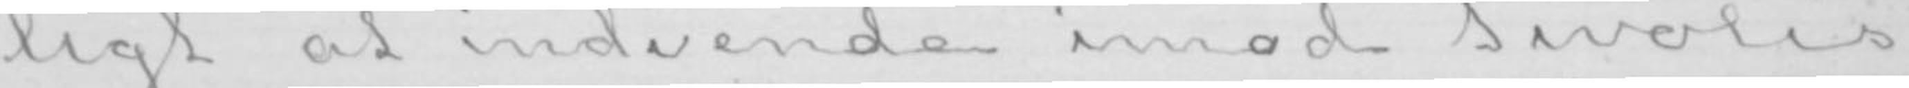ligt at indvende imod Tivolis
